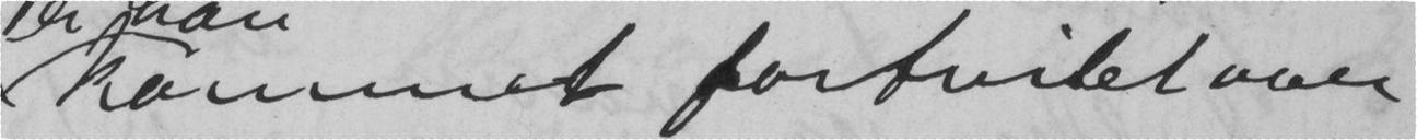kommet fortvilet over
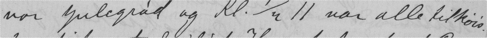var julegrød og Kl. 1/2 11 var alle tilkøis.
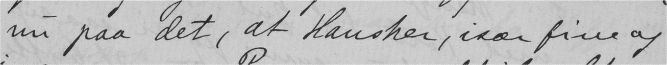nu paa det, at Hansker, især fine og
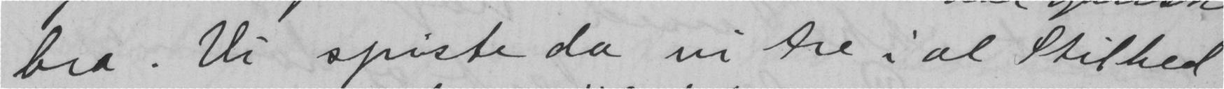bra. Vi spiste da vi tre i al Stilhed
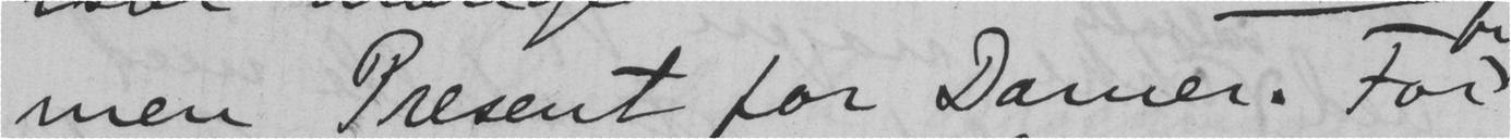men Present for Damer. For
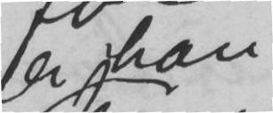er han
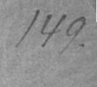149.
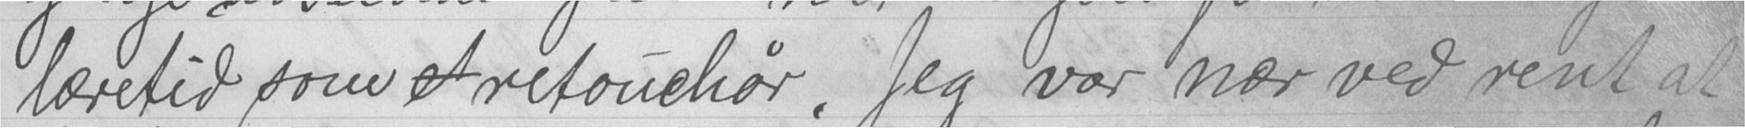læretid som et retouchør. Jeg var nær ved rent at
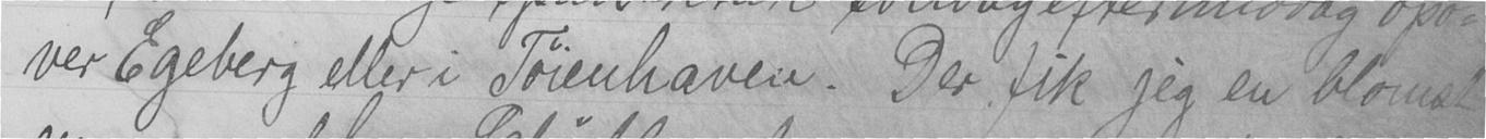ver Egeberg eller i Tøienhaven. Der fik jeg en blomst
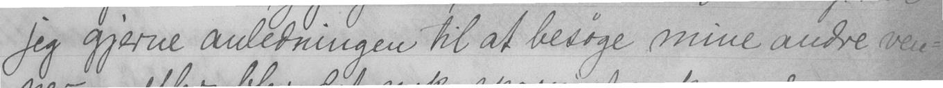jeg gjerne anledningen til at besøge mine andre ven-
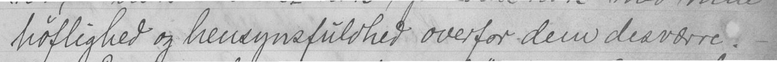høflighed og hensynsfuldhed overfor dem desværre. -
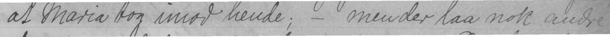at Maria tog imod hende; - men der laa nok andre
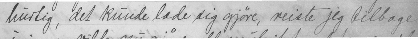hurtig, det kunde lade sig gjøre, reiste jeg tilbage
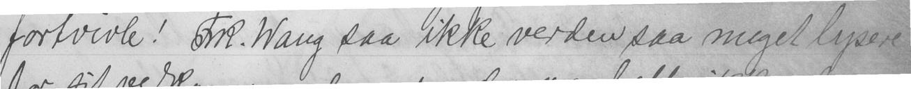fortvivle! Frk. Wang saa ikke verden saa meget lysere
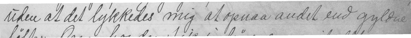uden at det lykkedes mig at opnaa andet end gyldne
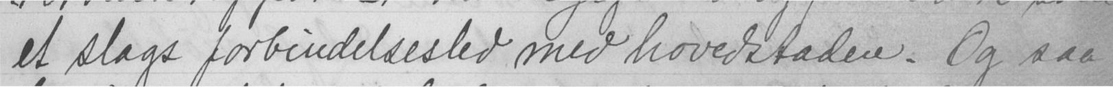et slagt forbindelsesled med hovedstaden. Og saa
